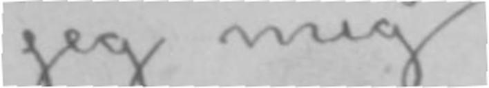jeg mig
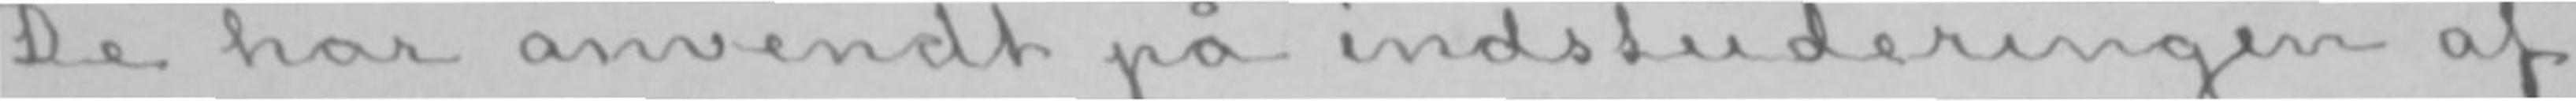De har anvendt på indstuderingen af
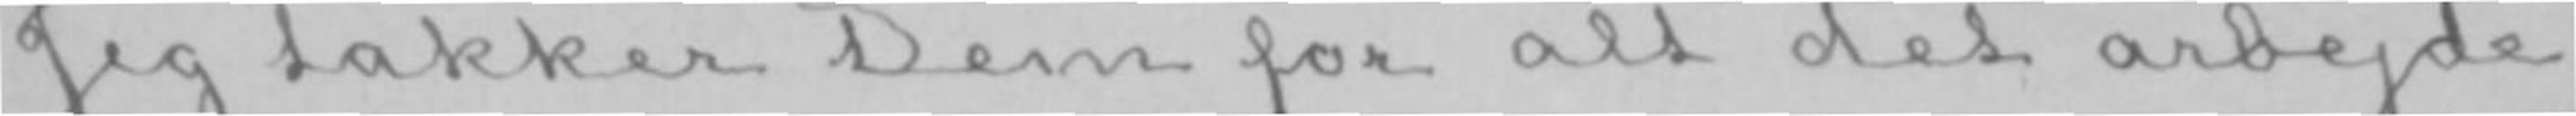Jeg takker Dem for alt det arbejde,
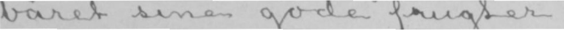båret sine gode frugter.
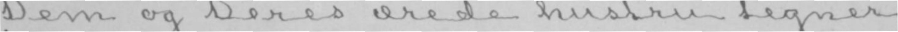Dem og Deres ærede hustru tegner
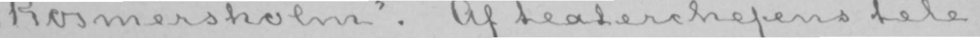«Rosmersholm». Af teaterchefens tele-
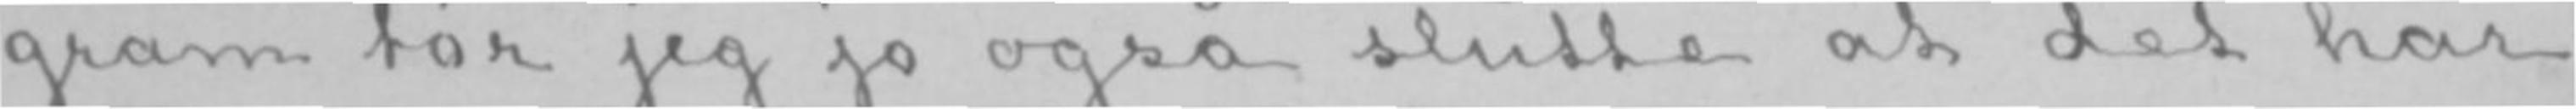gram tør jeg jo også slutte at det har
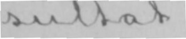sultat.
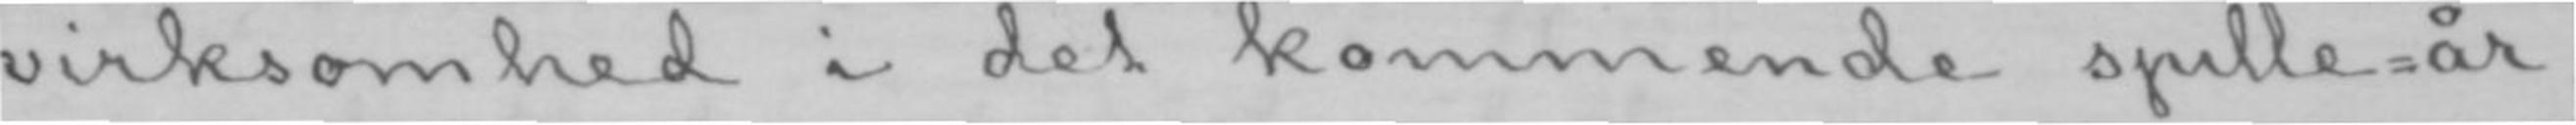virksomhed i det kommende spille-år;
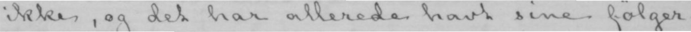ikke, og det har allerede havt sine følger.
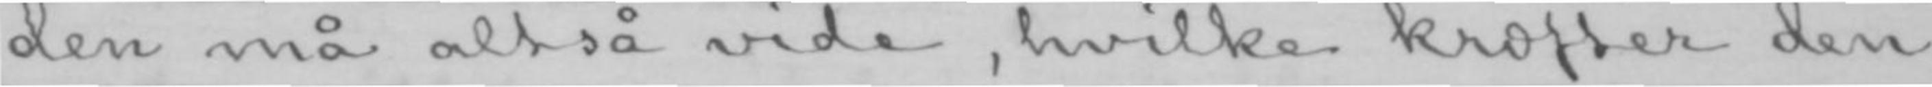den må altså vide, hvilke kræfter den
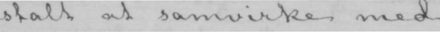stalt at samvirke med.
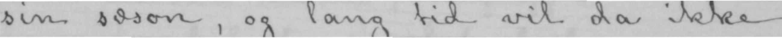sin sæson, og lang tid vil da ikke
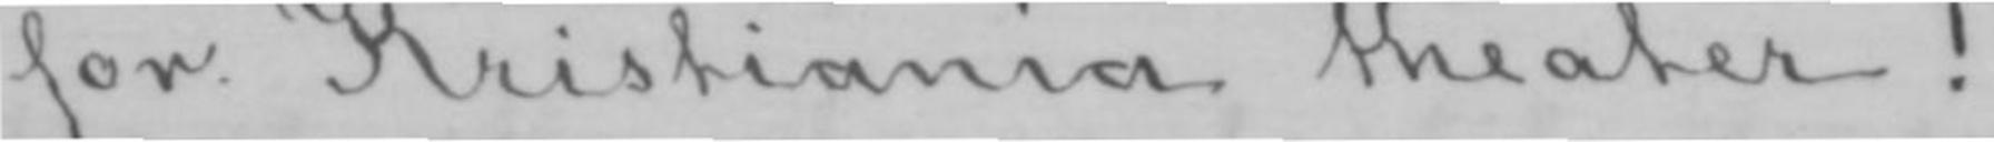for Kristiania theater!
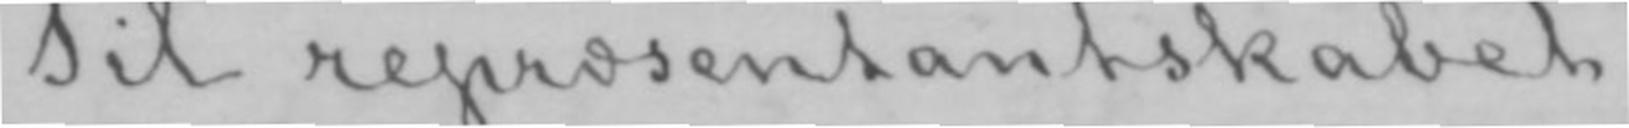Til repræsentantskabet
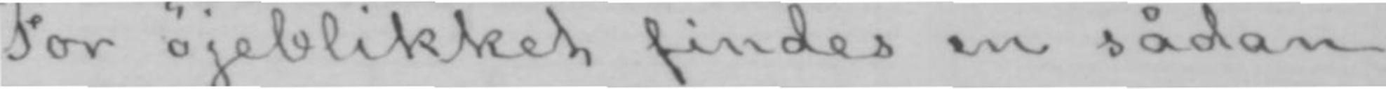For øjeblikket findes en sådan
